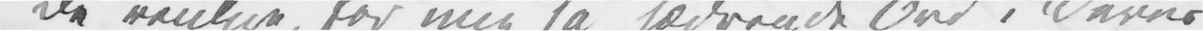De venlige, for mig så hædrende Ord i Deres
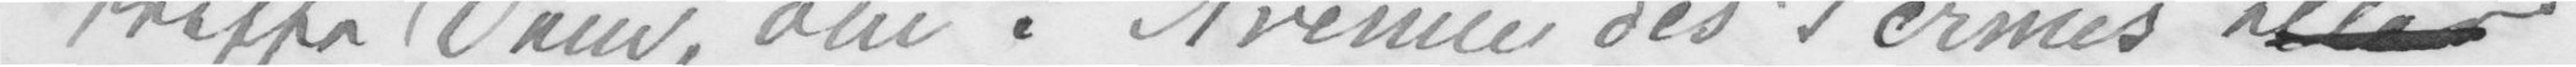treffe Dem, om i «Avenue des Termes» eller
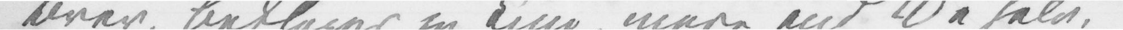Brev, beklager jeg kun, mere end De selv,
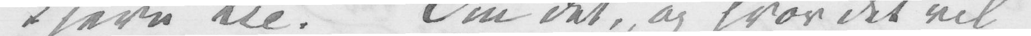Kjere Lie! Om det, og hvor det vil
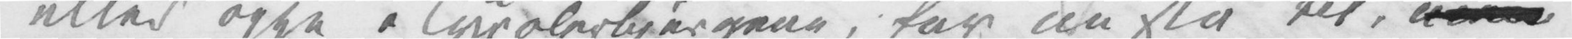eller oppe i Tyrolerbjergene, får nu stå til, men
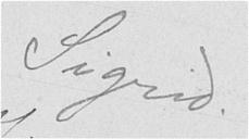Sigrid.
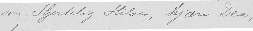son. Hjertelig Hilsen, kjære Dea,
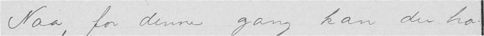Naa, for denne gang kan du ha-
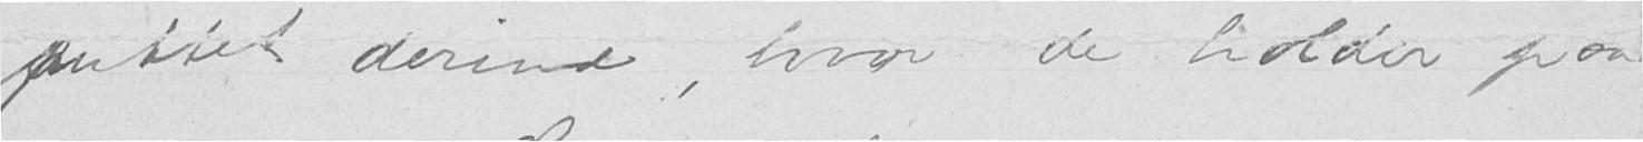putter derind, hvor de holder paa
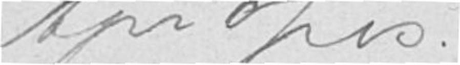Apropos.
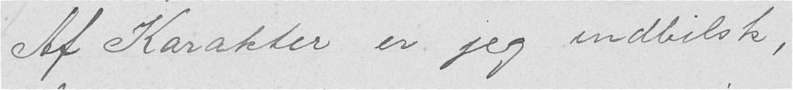Af Karakter er jeg indbilsk,
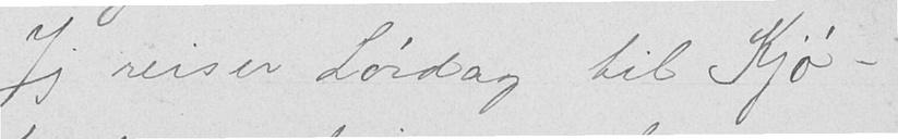Jeg reiser Lørdag til Kjø-
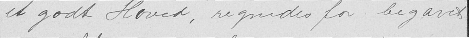et godt Hoved, regnedes for begavet
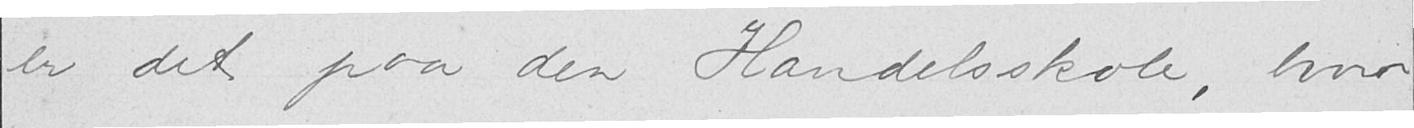er det paa den Handelsskole, hvor
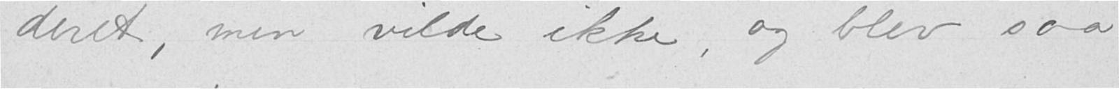deret, men vilde ikke, og blev saa
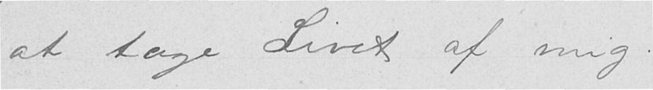at tage Livet af mig.
